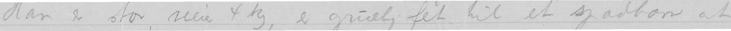Han er stor, veier 4 kg, er gruelig fet til et spædbarn at
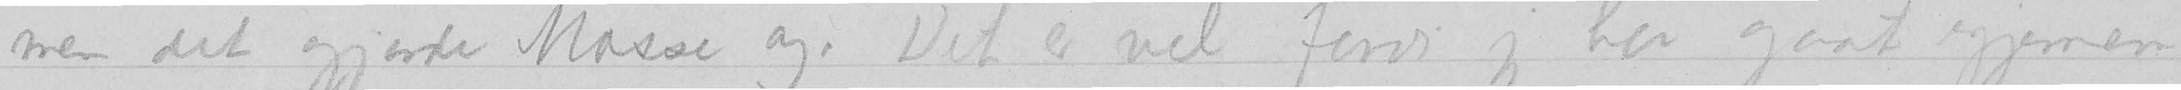men det gjorde Mosse og. Det er vel fordi jeg har gaat igjennem
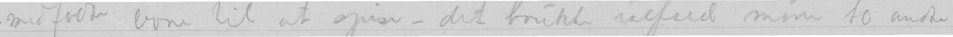medfødte evne til at spise – det brukte ialfald mine to andre
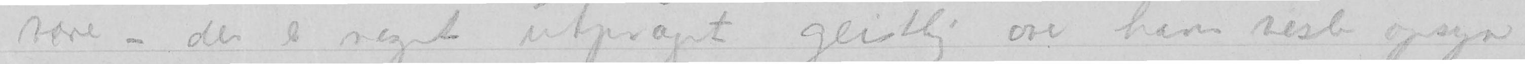være – der er noget utpræget geistlig over hans vesle opsyn
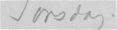Torsdag
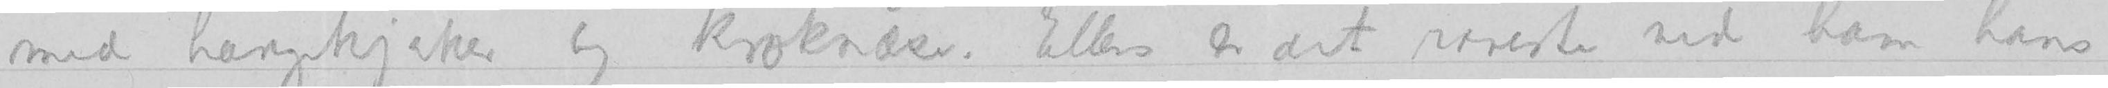med hængekjaker og kroknæse. Ellers er det rareste ved ham hans
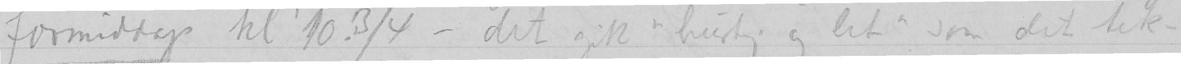formiddags kl’ 10.3/4 – det gik «hurtig og let» som det tek-
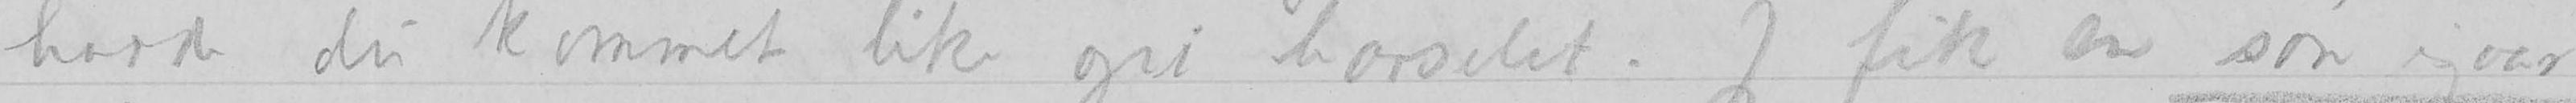hadde du kommet like opi barselet. Jeg fik en søn igaar
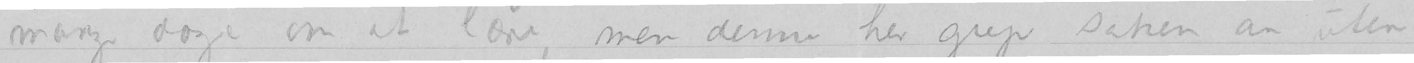mange dager om at lære, men denne her grep saken an uten
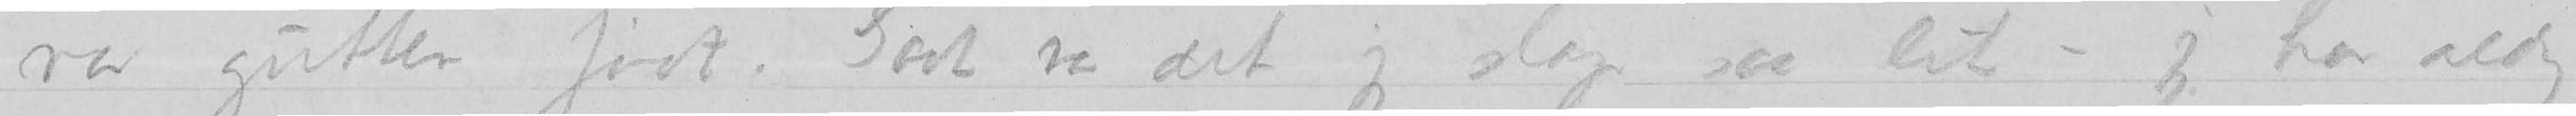var gutten født. Godt var det jeg slap saa let – jeg har aldrig
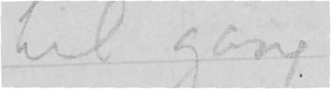til gang –
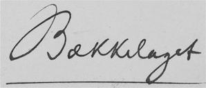Bækkelaget
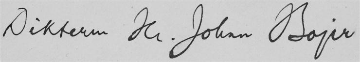Dikteren Hr. Johan Bojer
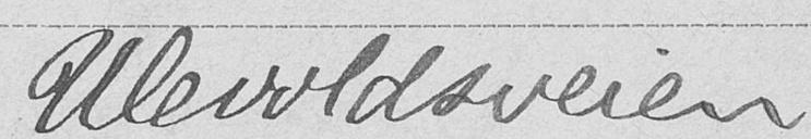Ulevoldsveien
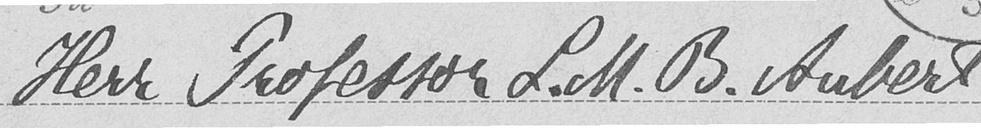Herr Professor L. M. B. Aubert
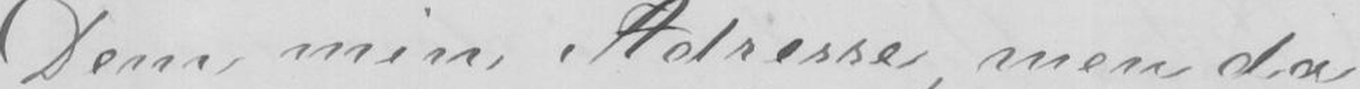Dem min Adresse, men da
